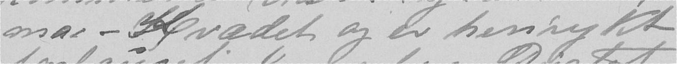ma-Kvædet og er henrykt
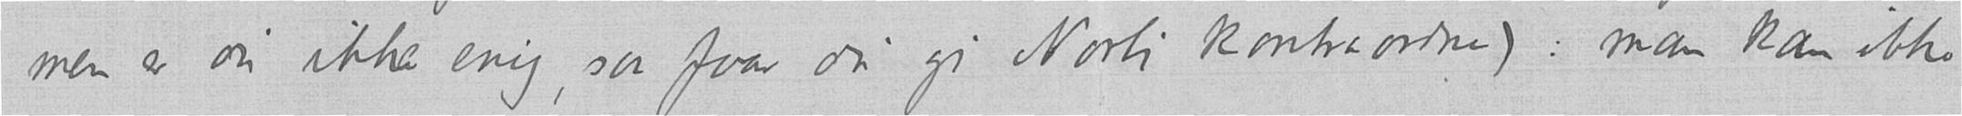men er du ikke enig, saa faar du gi Norli kontraordne): man kan ikke
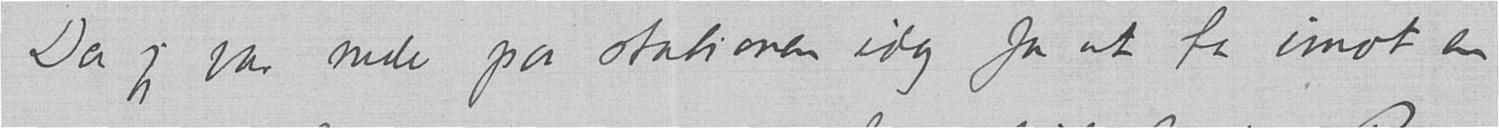Da jeg var nede paa stationen idag for at ta imot en
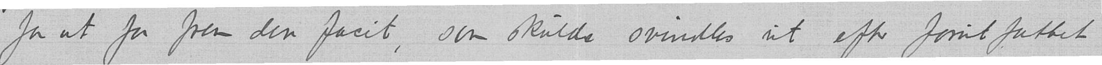for at faa frem den facit, som skulde svindles ut efter forutfattet
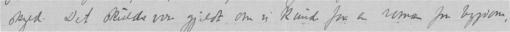skyld. Det skulde være gjildt om vi kunde faa en roman fra bygdom,
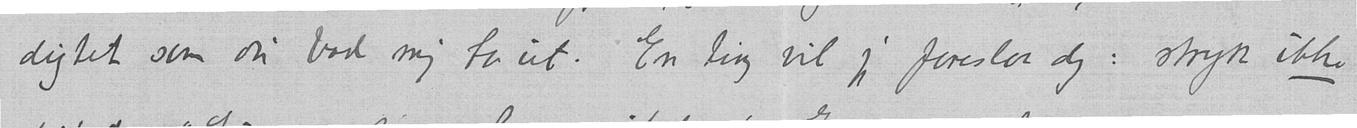digtet som du bad mig ta ut. En ting vil jeg foreslaa dig: stryk ikke
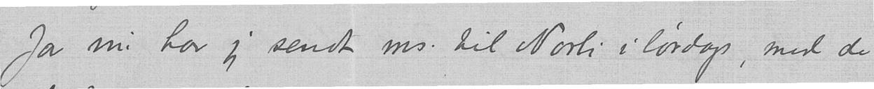Ja nu har jeg sendt ms. til Norli i lørdags, med de
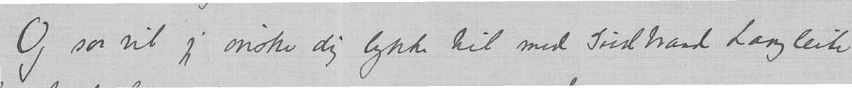Og saa vil jeg ønske dig lykke til med Gudbrand Langleite
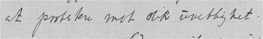at protestere mot slik uvettighet.
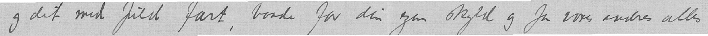og det med fuld fart, baade for din egen skyld og for vores andres alles
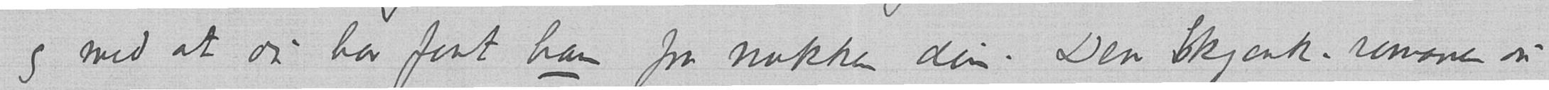og med at du har faat ham fra nakken din. Den skjenk-romanen du
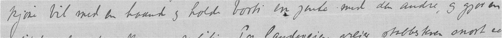kjøre bil med en haand og holde borti en jente med den andre, og gjør en
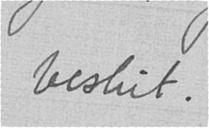beslut.
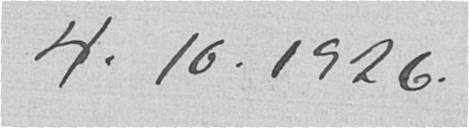4.10.1926.
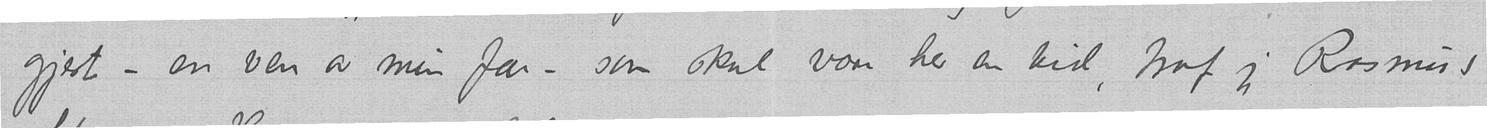gjest – en ven av min far – som skal være her en tid, traf jeg Rasmus
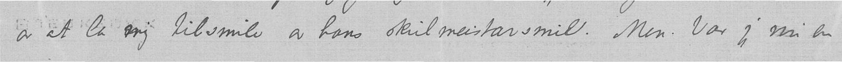av at la mig tilsmile av hans skulmeistarsmil. Men. Var jeg nu en
